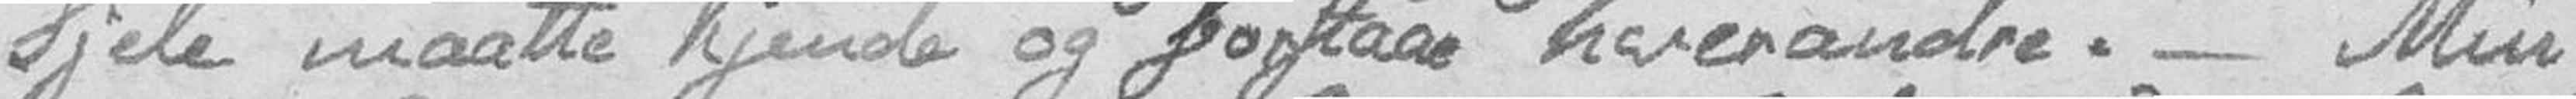Sjele maatte kjende og forstaae hverandre. – Min
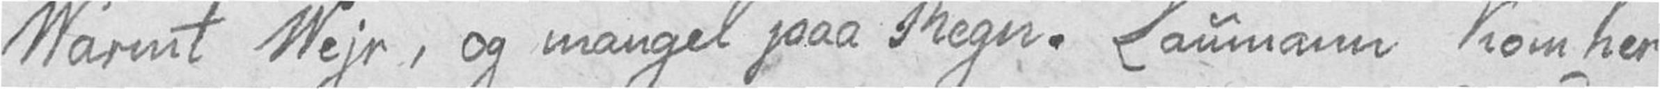Varmt Vejr, og mangel paa Regn. Laumann kom her
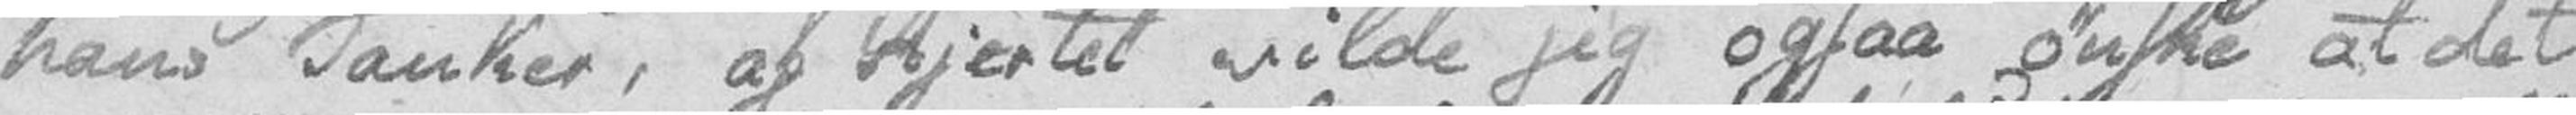hans Tanker, af Hjertet vilde jeg ogsaa ønske at det
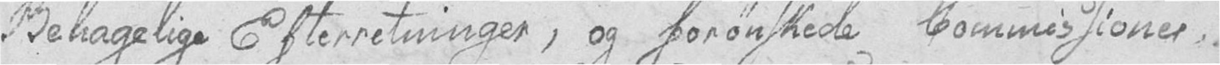Behagelige Efterretninger, og forønskede Commissioner
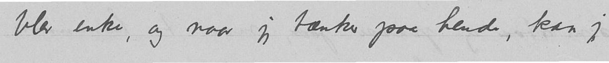blev enke, og naar jeg tænker paa hende, kan jeg
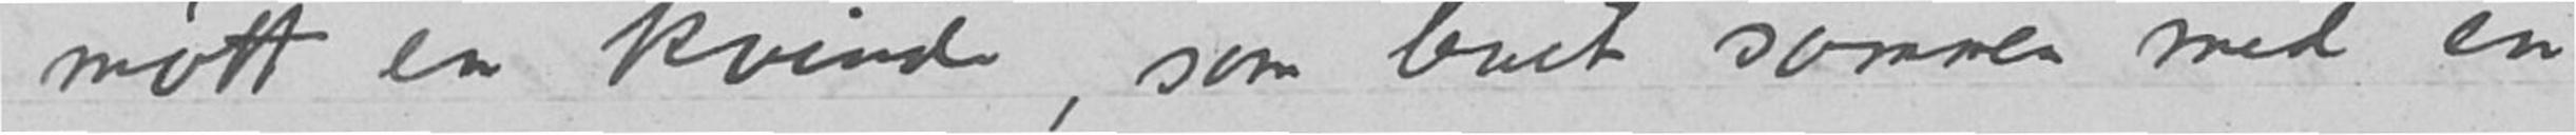møtt en kvinde, som levet sammen med en
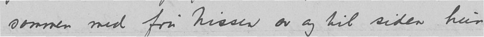sammen med fru Nissen av og til siden hun
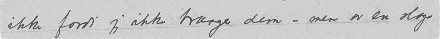ikke fordi jeg ikke trænger dem – men av en slags
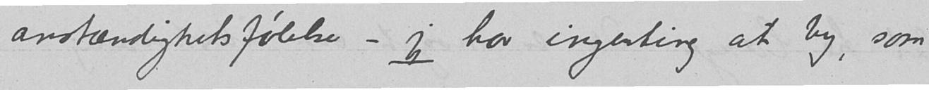anstændighetsfølelse – jeg har ingenting at by, som
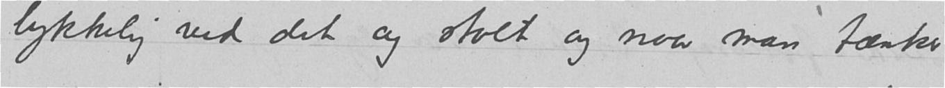lykkelig ved det og stolt og naar man tænker
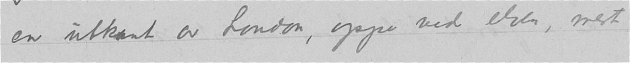en utkant av London, oppe ved elven, mest
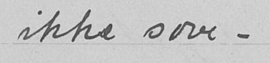ikke sove - .
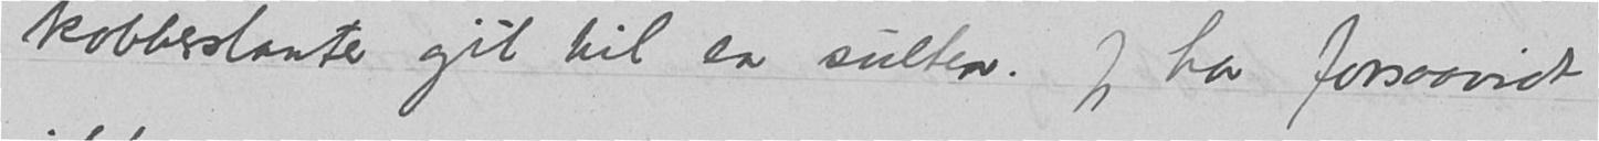kobberslanter git til en sulten. Jeg har forsaavidt
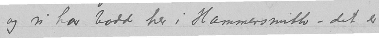og vi har bodd her i Hammersmith – det er
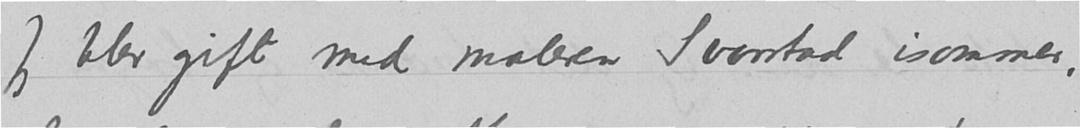Jeg blev gift med maleren Svarstad isommer,
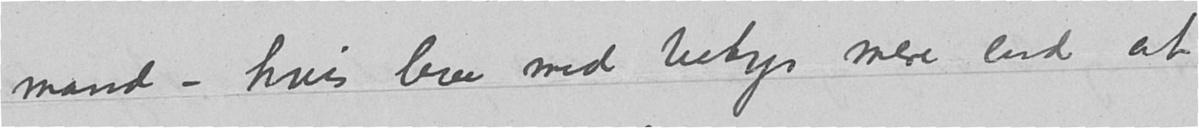mand – hvis leve med betyr mere end at
